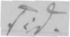Tid.
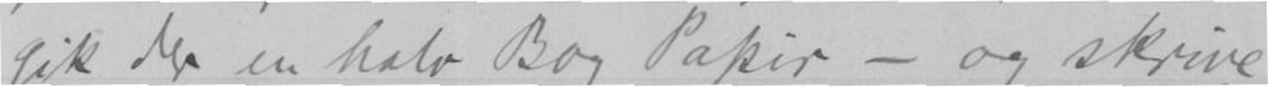gik der en halv Bog Papir - og skrive
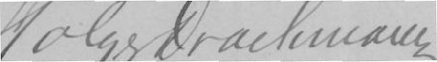Holger Drachmann
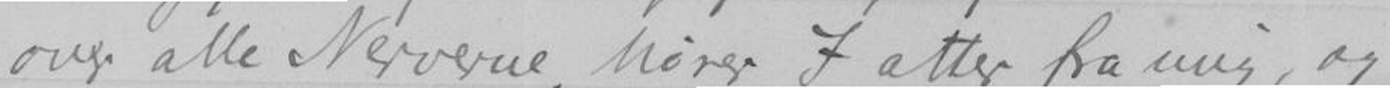over alle Nerverne, hører I atter fra mig, og
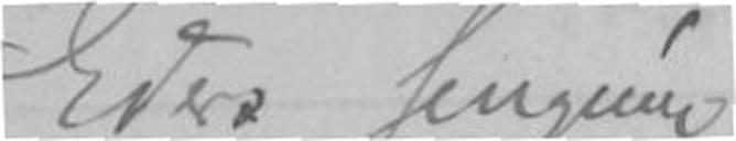Eders hengivne
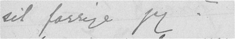sit forrige jeg?
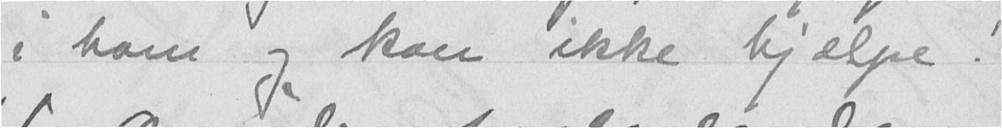i ham og kan ikke hjælpe!
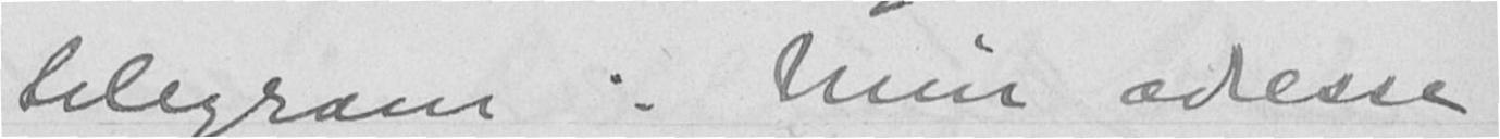telegram: min adresse
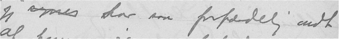jeg synes har saa forfærdelig ondt
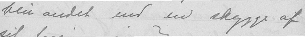blir andet end en skygge af
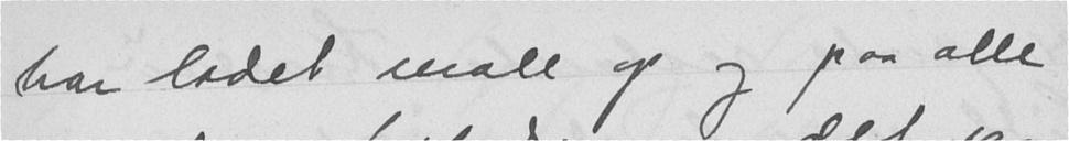har ladet male op og paa alle
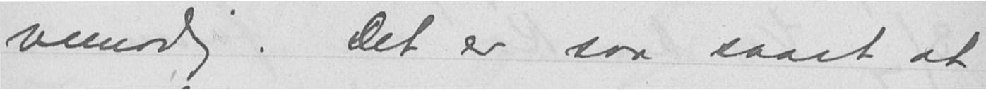vemodig. Det er saa saart at
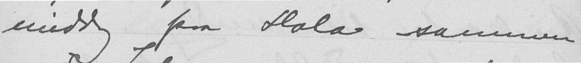middag paa Hola sammen
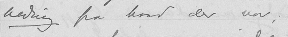bedring fra hvad der var;
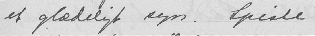et glædeligt syn. Spiste
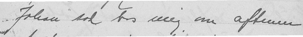Johan sad hos mig om aftenen
